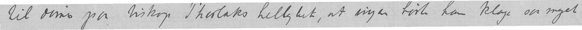til dømer paa biskop Thorlaks hellighet, at ingen hørte ham klage saa meget
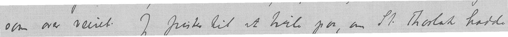som over veiret. Jeg fristes til at tvile paa, om St. Thorlak hadde
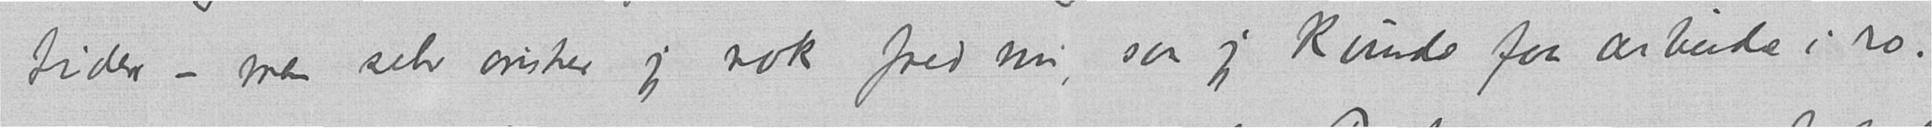tider – men selv ønsker jeg nok fred nu, saa jeg kunde faa arbeide i ro.
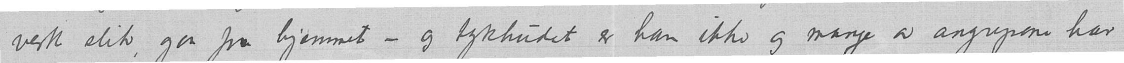verk slik, gaa fra hjemmet – og tykhudet er han ikke og mange av angrepene har
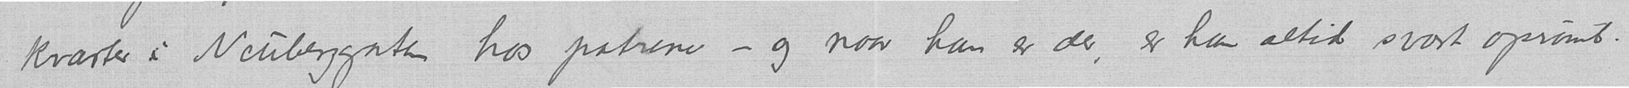kvarter i Neuberggaten hos patrene – og naar han er der, er han altid svært oprømt.
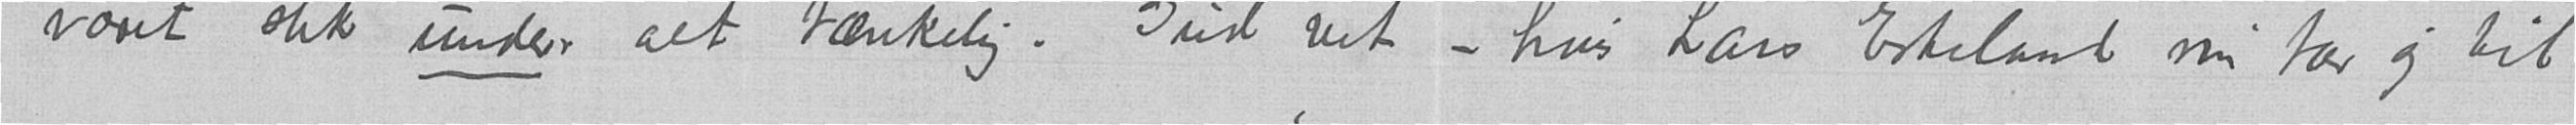været slik under alt tænkelig. Gud vet – hvis Lars Eskeland nu tar sig til
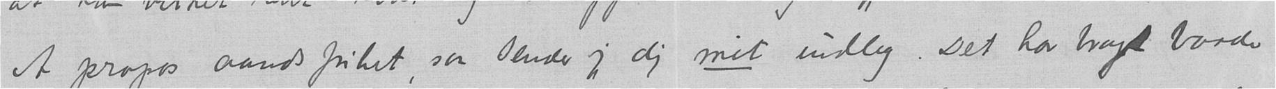A propos aandsfrhet saa sender jeg dig mit indleg. Det har bragt baade
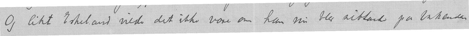Og likt Eskeland vilde det ikke være om han nu blev sittende paa bakenden
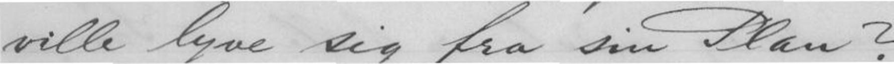ville lyve sig fra sin Plan?
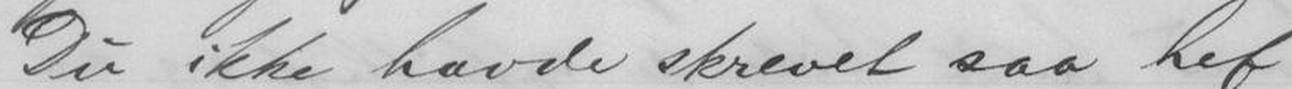Du ikke havde skrevet saa hef-
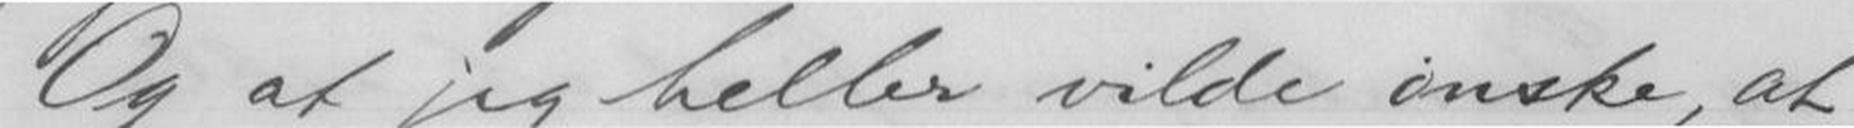Og at jeg heller vilde ønske, at
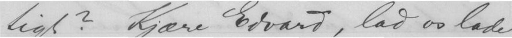tigt? Kjære Edvard, lad os lade
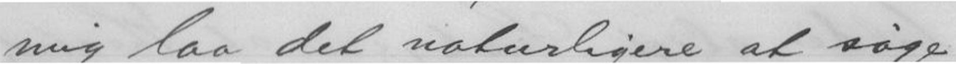mig laa det naturligere at søge
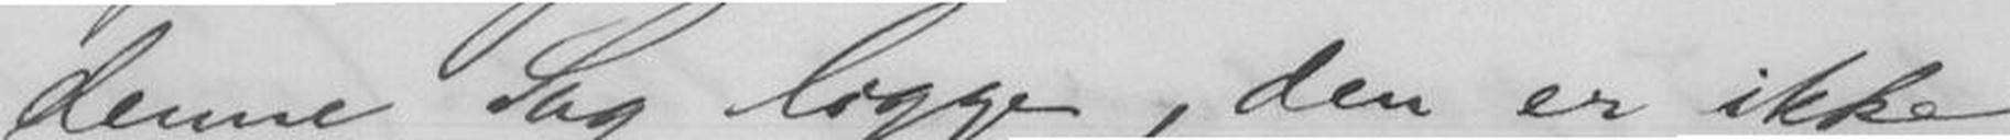denne Sag ligge, den er ikke
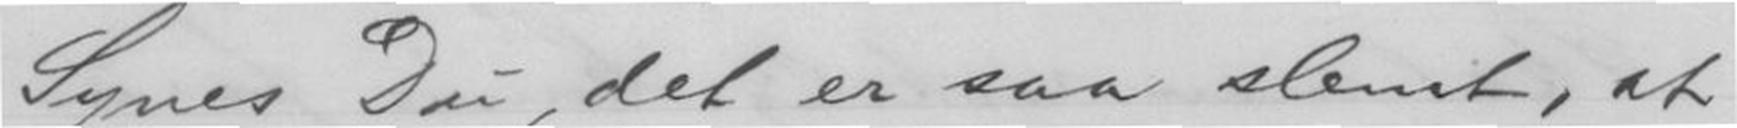Synes Du, det er saa slemt, at
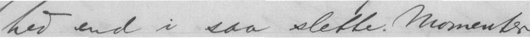hed end i saa slette Momenter.
